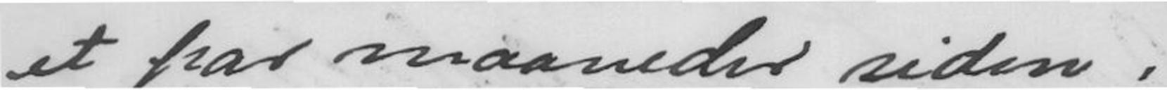et par maaneder siden,
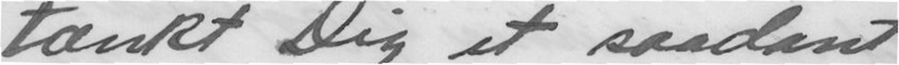tænkt Dig et saadant
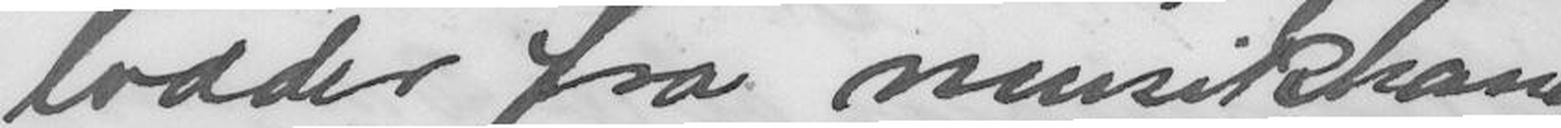lodder fra musikhandlen.
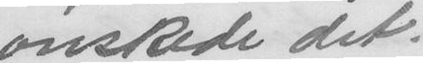ønskede det.
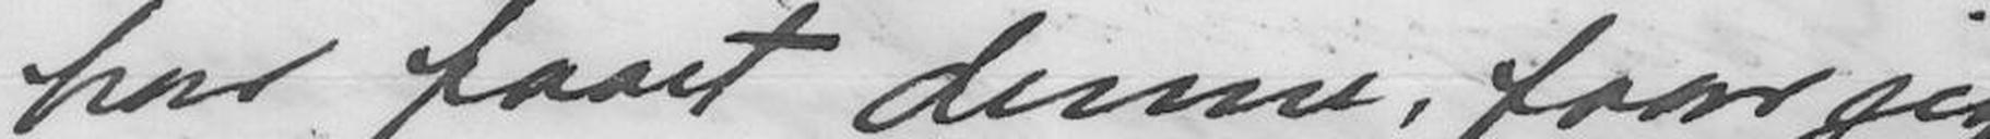har faaet denne, faar jeg
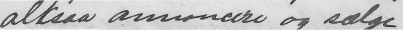altsaa annoncere og selge
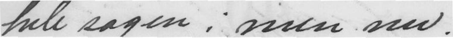hele sagen; men nu.
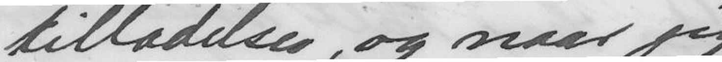tilladelse, og naar jeg
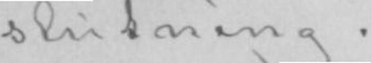slutning.
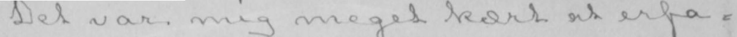Det var mig meget kært at erfa-
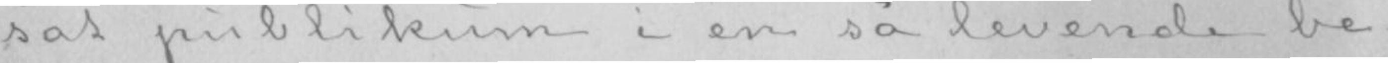sat publikum i en så levende be-
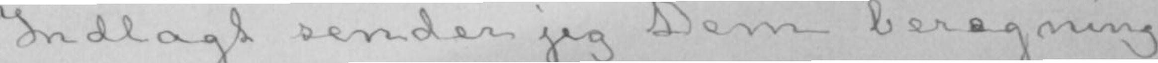Indlagt sender jeg Dem beregning
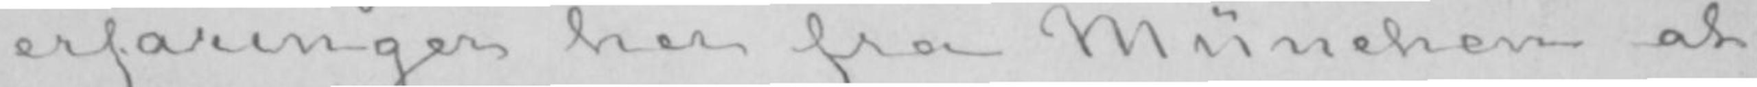erfaringer her fra München at
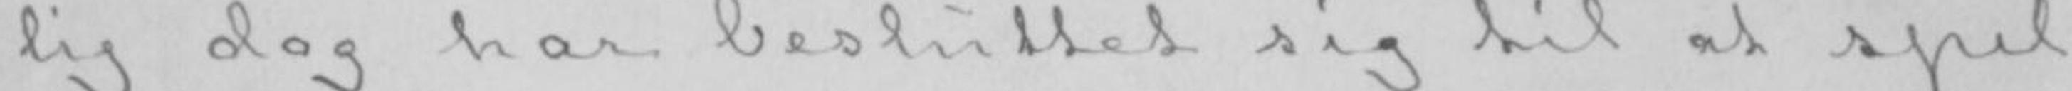lig dog har besluttet sig til at spil-
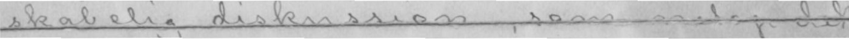skabelig diskussion, som netop dette.
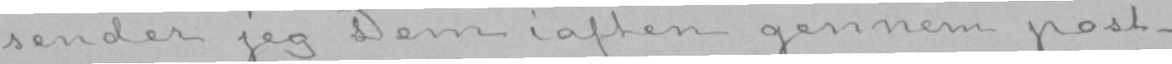sender jeg Dem iaften gennem post-
